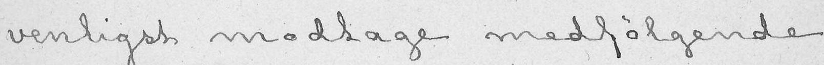venligst modtage medfølgende
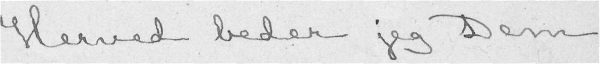Herved beder jeg Dem
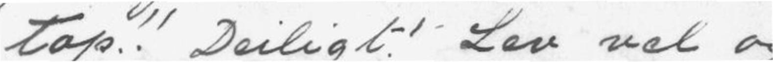top!! Deiligt! Lev vel og
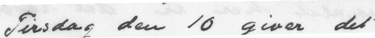Tirsdag den 10 giver det
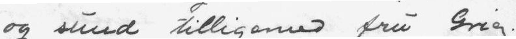og sund tilligemend fru Grieg.
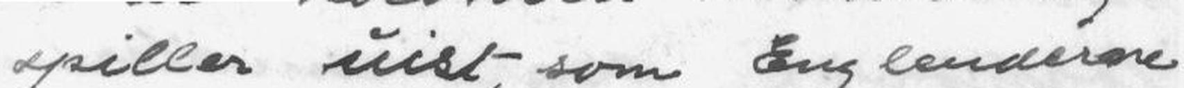spiller uist, som Englenderne
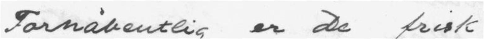Forhåbentlig er De frisk
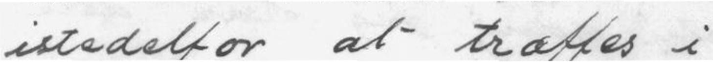istedetfor at træffes i
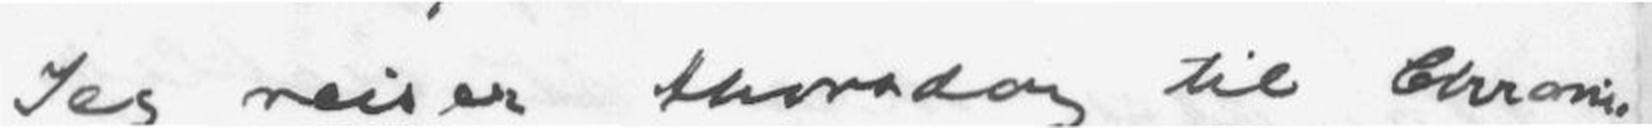Jeg reiser thorsdag til Chrania.
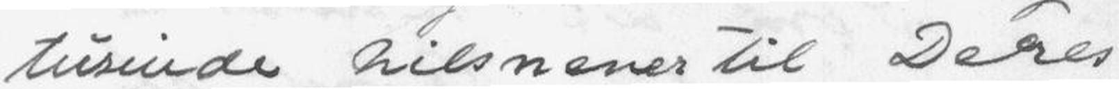tusinde hilsnener til Deres
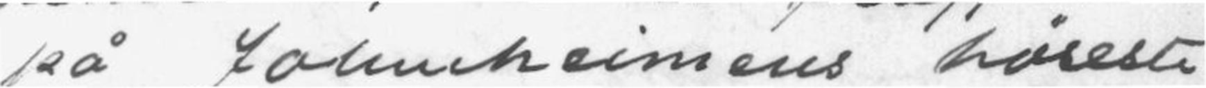på Jotunheimens høieste
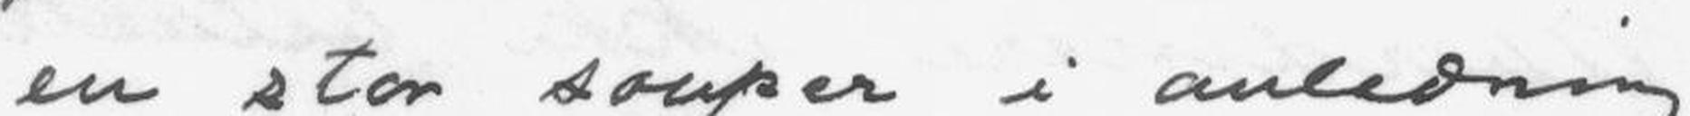en stor souper i anledning
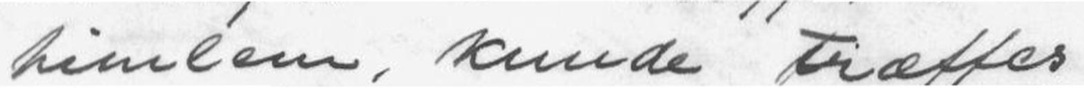himlen, kunde træffes
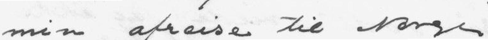min afreise til Norge
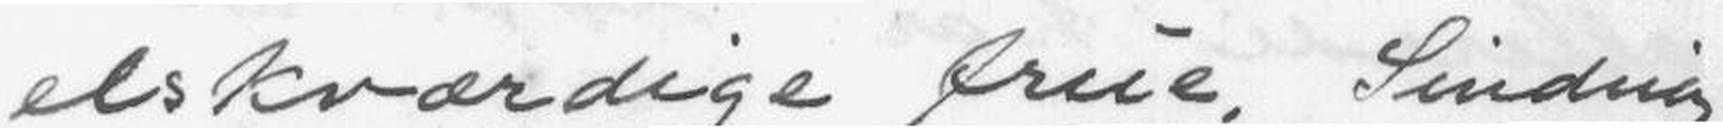elskværdige frue, Sinding
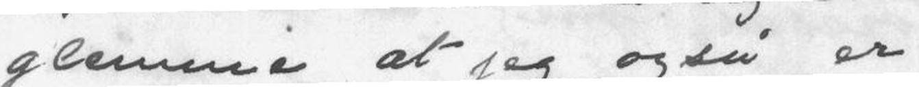glemme at jeg også er
